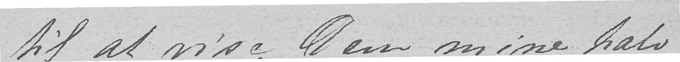til at vise Dem mine halv-
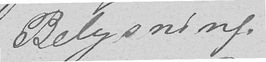Belysnings.
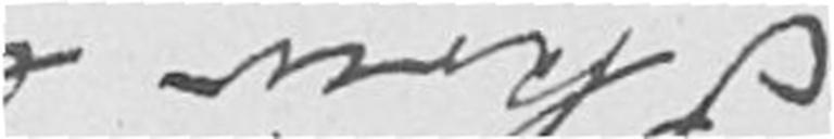Skriv endelig snart, og fortæl mig
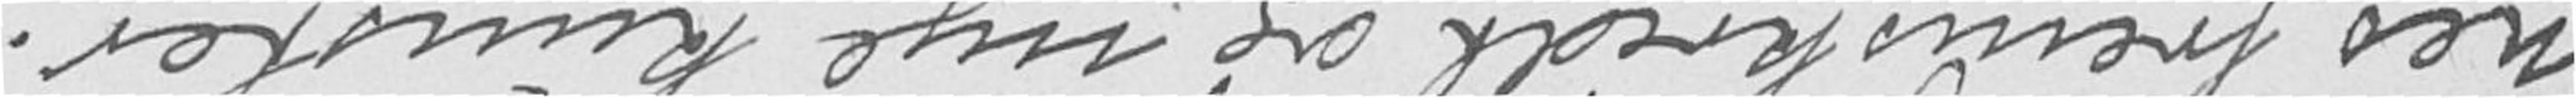nes fremskridt og nye kunster.
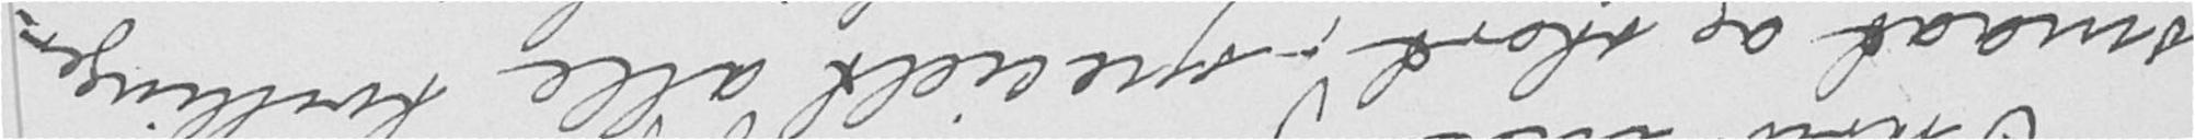smaat og stort, - specielt alle tvillinger-
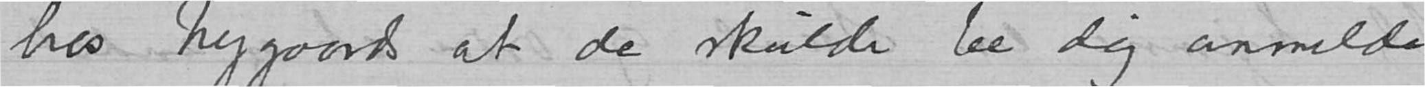hos Nygaards at de skulde be dig anmelde
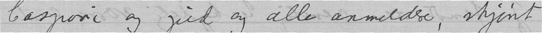Caspari og gud og alle anmeldere, skjønt
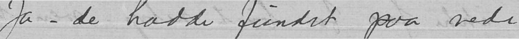Ja – de hadde fundet paa nede
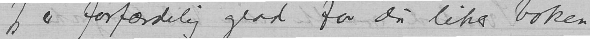Jeg er forfærdelig glad for du liker boken
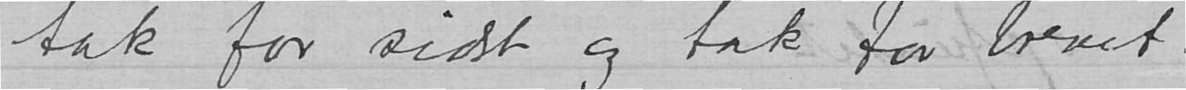tak for sidst og tak for brevet.
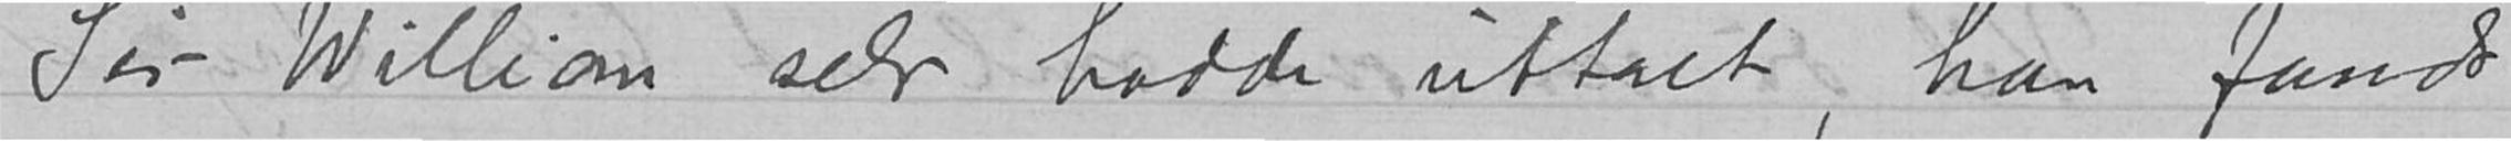Sir William selv hadde uttalt, han fandt
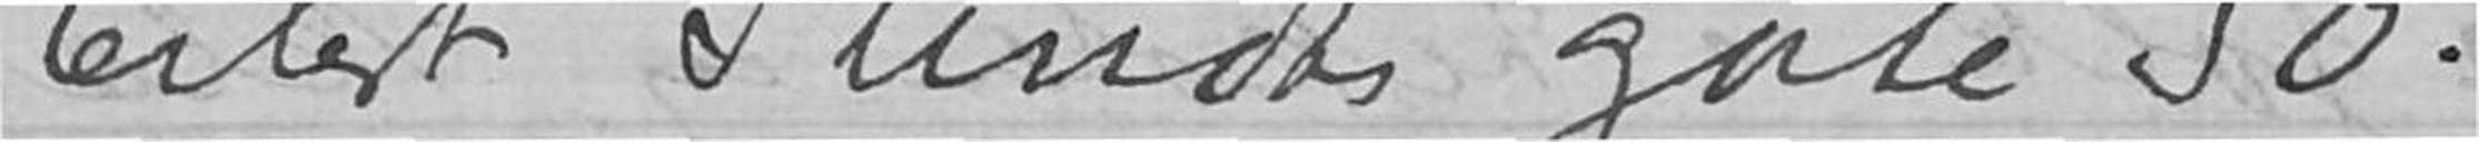Eilert Sundts gate 50.
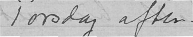Torsdag aften.
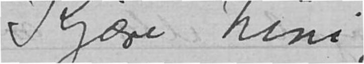Kjære Nini,
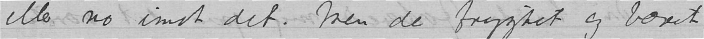eller no imot det. Men de frygtet og bævet
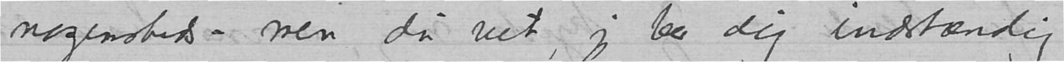nogensteds – men du vet, jeg ber dig indstændig
